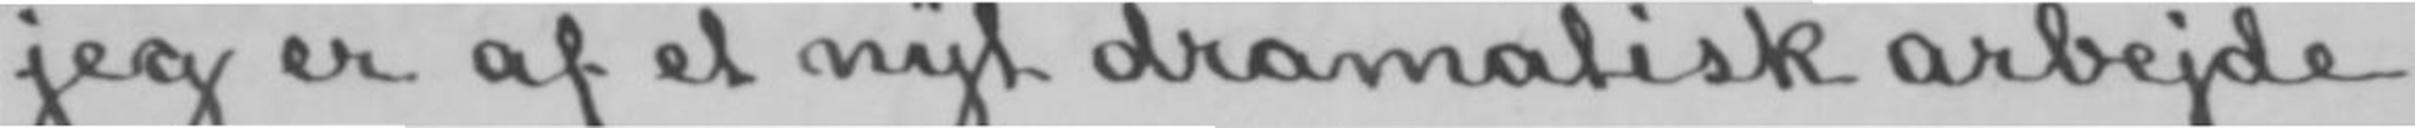jeg er af et nyt dramatisk arbejde
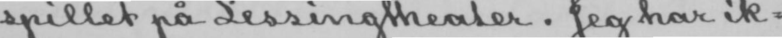spillet på Lessingtheater. Jeg har ik-
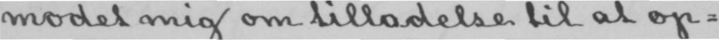modet mig om tilladelse til at op-
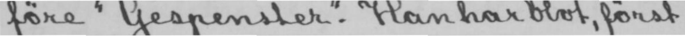føre «Gespenster». Han har blot, først
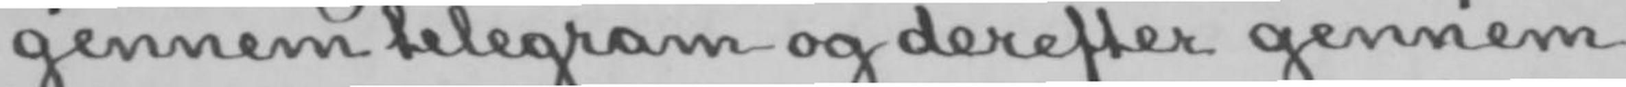gennem telegram og derefter gennem
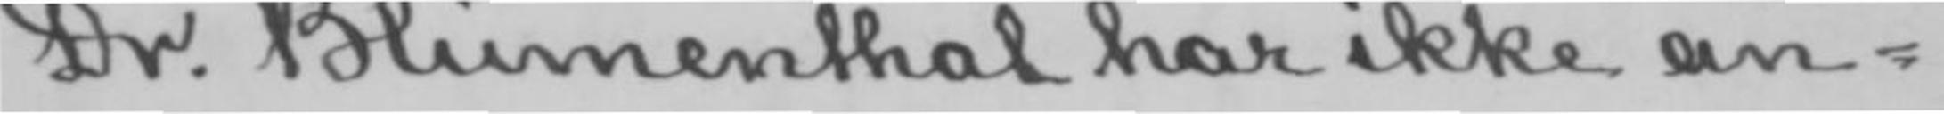Dr. Blumenthal har ikke an-
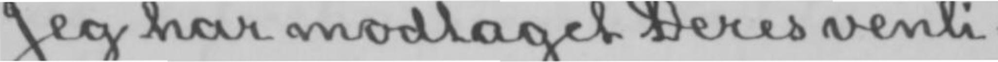Jeg har modtaget Deres venli-
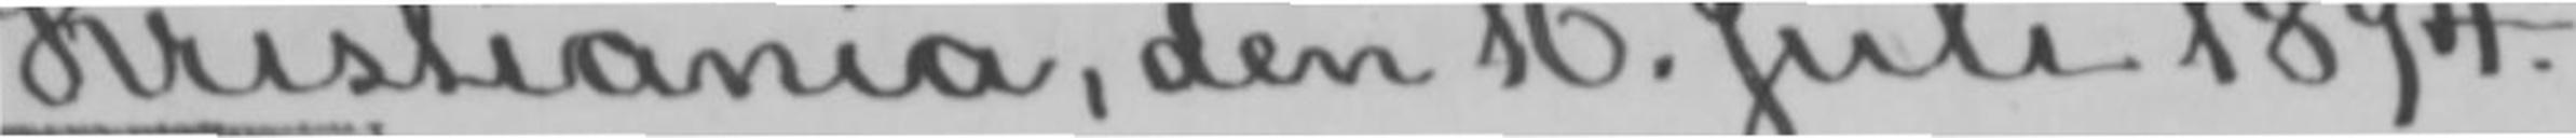Kristiania, den 16. Juli 1894.
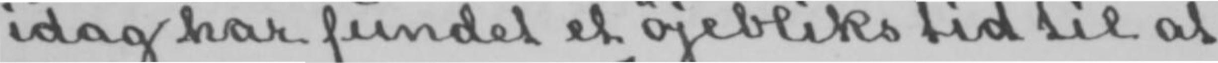idag har fundet et øjebliks tid til at
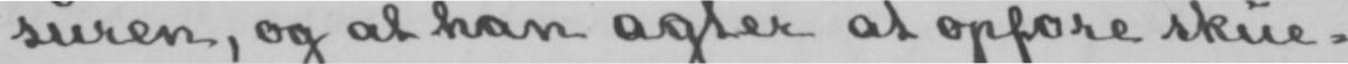suren, og at han agter at opføre skue-
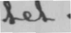tet.
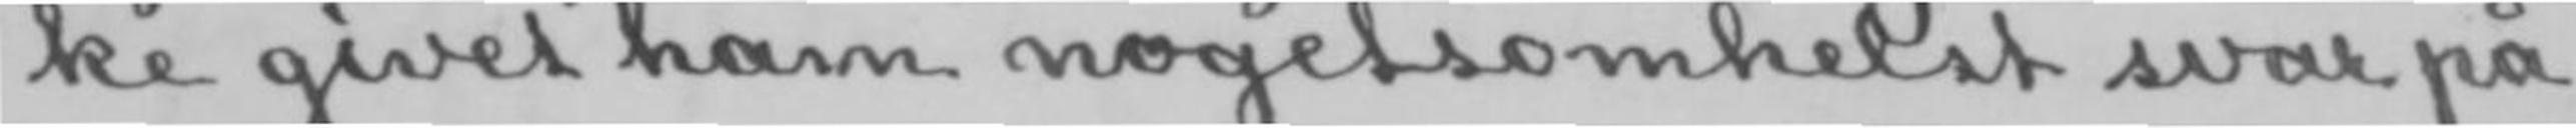ke givet ham nogetsomhelst svar på
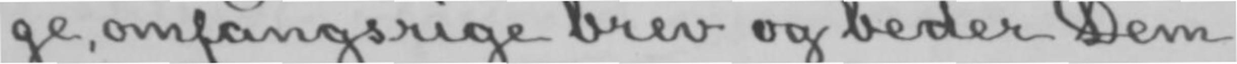ge, omfangsrige brev og beder Dem
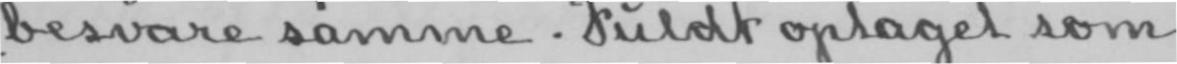besvare samme. Fuldt optaget som
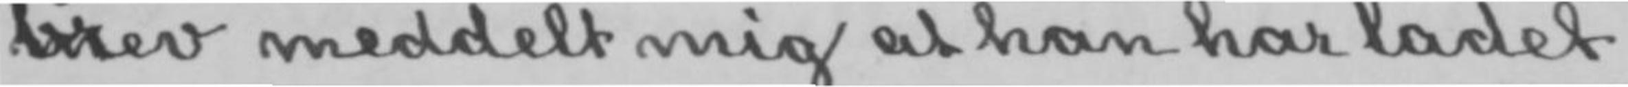brev meddelt mig at han har ladet
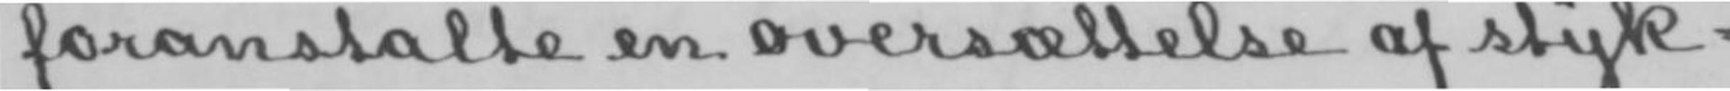foranstalte en oversættelse af styk-
